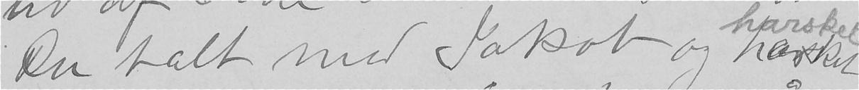du talt med Jakob og harsket
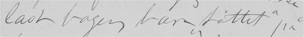læst bogen, bare "tittet" på
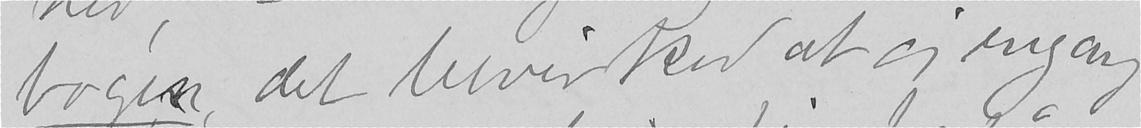bogen, det bevirked at jeg engang
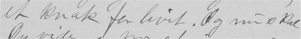et knæk for livet. Og nu skal
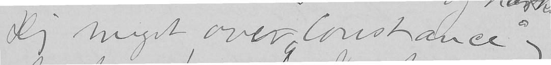Dig meget over "Constance",
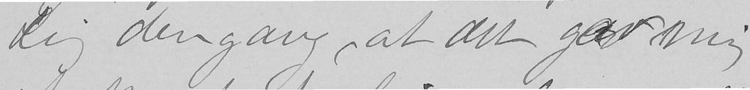Dig dengang, at det gav mig
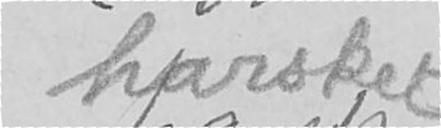harsket
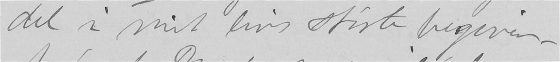del i mit livs største begiven-
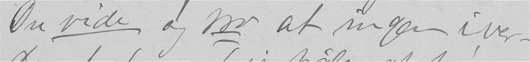Du vide og tro at ingen iver-
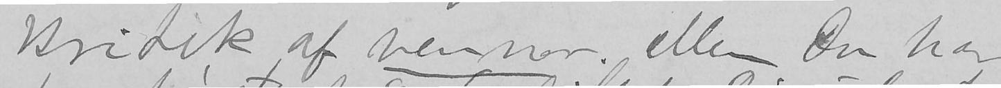kritik af venner. Men Du har
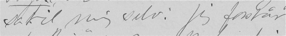sa til mig selv: Jeg forstår
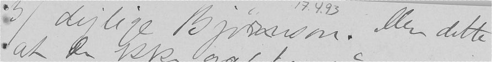3/ dejlige Bjørnson. Men dette
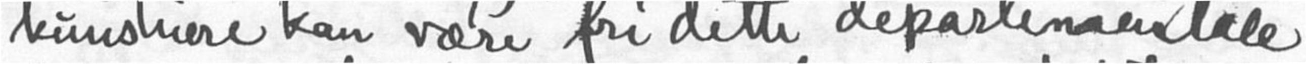kunstnere kan være fri dette departementale
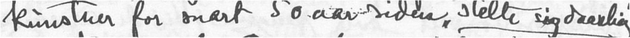kunstner for snart 50 aar siden "stelte sig daarlig"
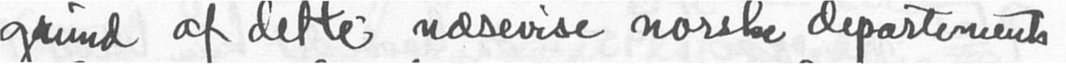grund af dette næsevise norske departements
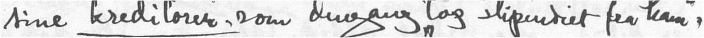sine kreditorer, som dengang "tog stipendiet fra ham"
